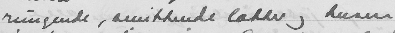rungende, smittende latter og tusen
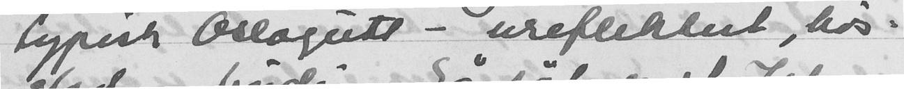typisk Oslogutt - ureflektert, hos-
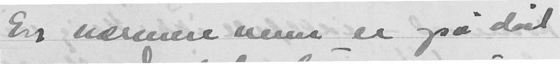En nærmere venn er også død
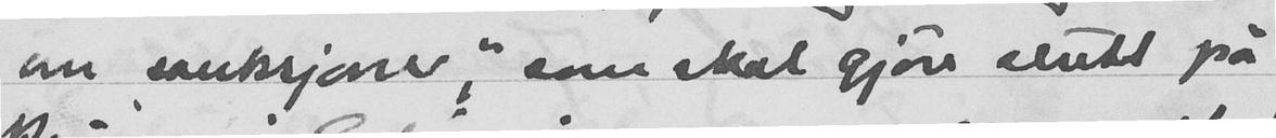om sanksjoner, som skal gjøre slutt på
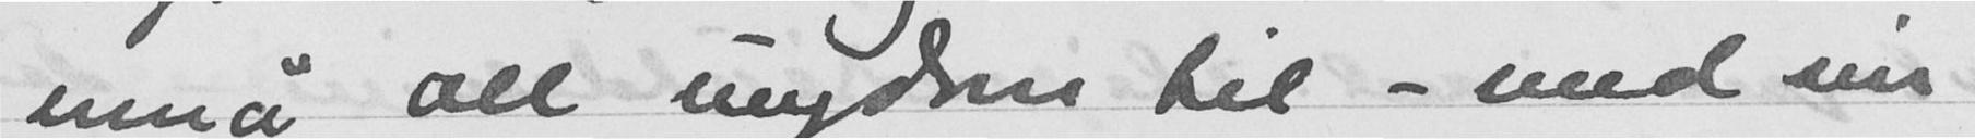ennå all ungdom til - med sin
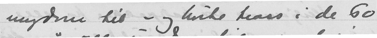ungdom til - og brukte tross i de 60
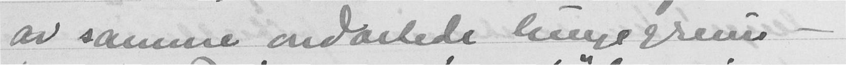av samme ondartede lungegrums -
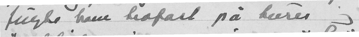fulgte ham trofast på turer og
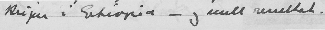krigen i Etiopia - og null resultat.
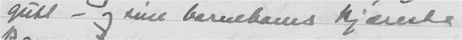gutt - og sine barnebarns kjæreste
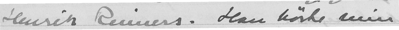Henrik Reimers. Han hørte min
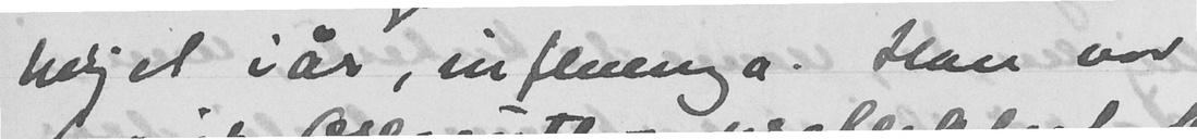herjet iår, influensa. Han var
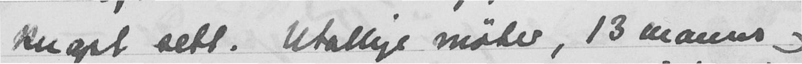knapt sett. Utallige møter, 13 manns og
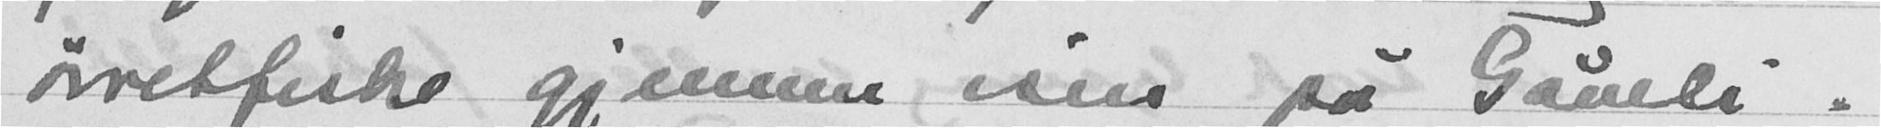ørretfiske gjennom isen på Gåneli-
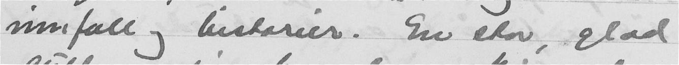infall og historier. En stor, glad
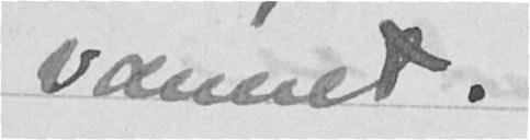vannet.
